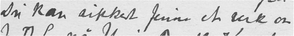Du kan sikkert finne et verk om
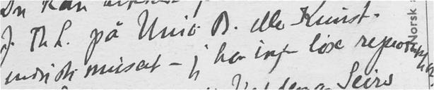industrimuseet – jeg har ingen løse reproduktioner.
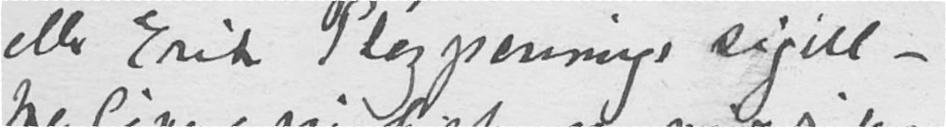eller Erik Plogpennings sigill -
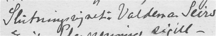Slutningsvignet: Valdemar Seiers
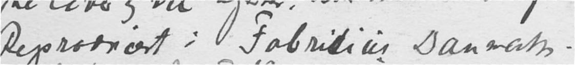Reprodusert i Fabricius Danmarks-
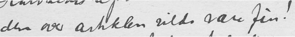dem over artiklen vilde være fin!
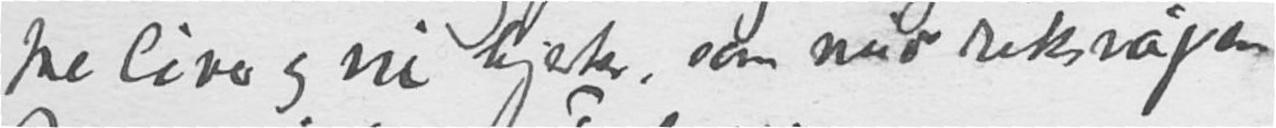tre løver og ni hjerter, som nu er riksvåpen
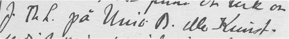J. Th. L. pa Univ.B. eller Kunst-
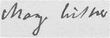Mange hilsener
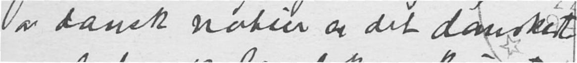av dansk natur er det danskeste
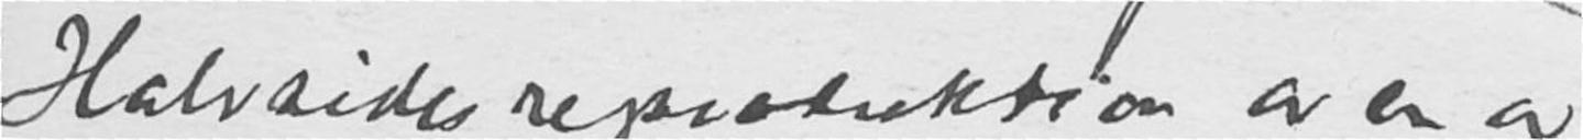Halvsides reproduktion av en av
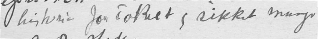historie for Folket og sikkert mange
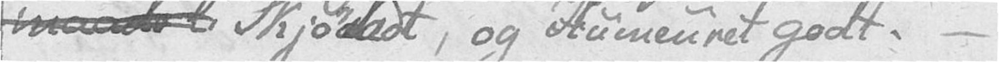maadel Skjøndt, og Humeuret godt. –
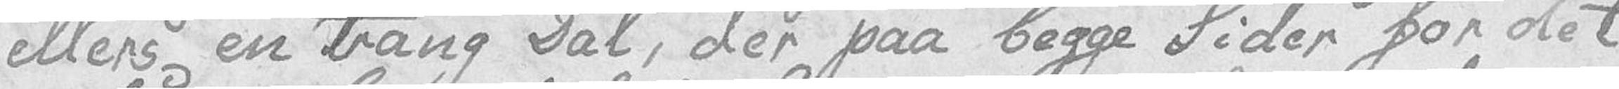ellers en trang Dal, der paa begge Sider for det
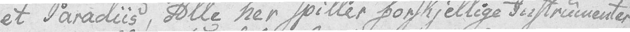et Paradiis, Alle her spiller forskjellige Instrumenter
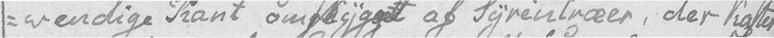-vendige Kant omskygget af Syrintræer, der kaster
